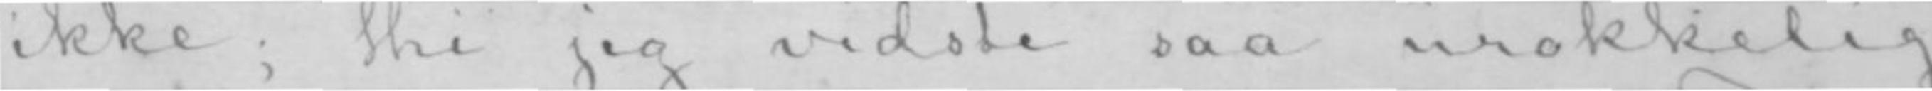ikke; thi jeg vidste saa urokkelig
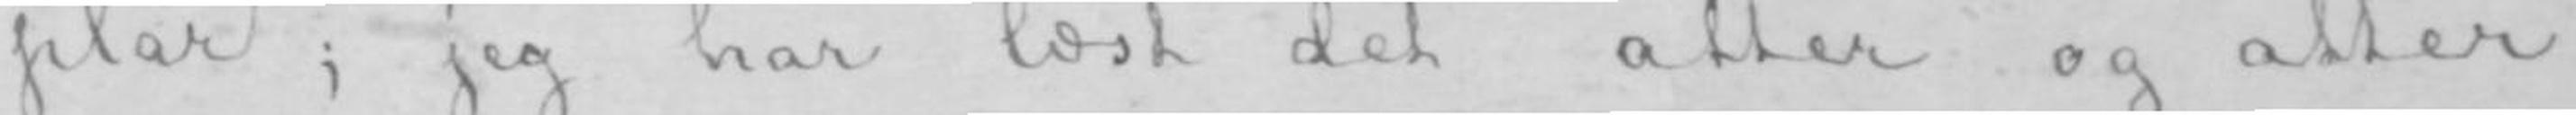plar; jeg har læst det atter og atter,
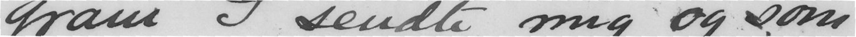gram I sendte mig og som
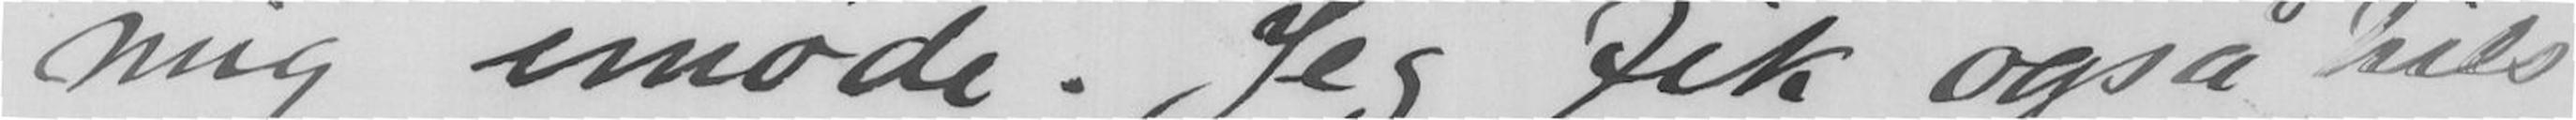mig imøde. Jeg fik også hils
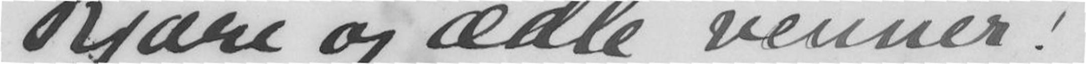Kjære og ædle venner !
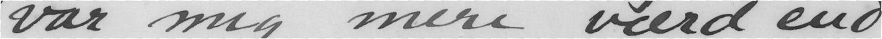var mig mere værd end
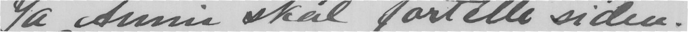Ja Annie skal fortelle siden.
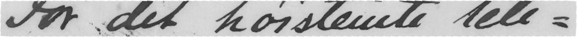For det høistemte tele-
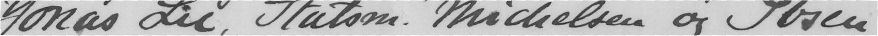Jonar Lie, Statsm. Michelsen og Ibsen
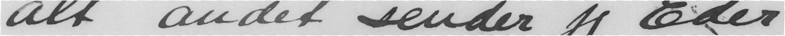alt andet sender jeg Eder
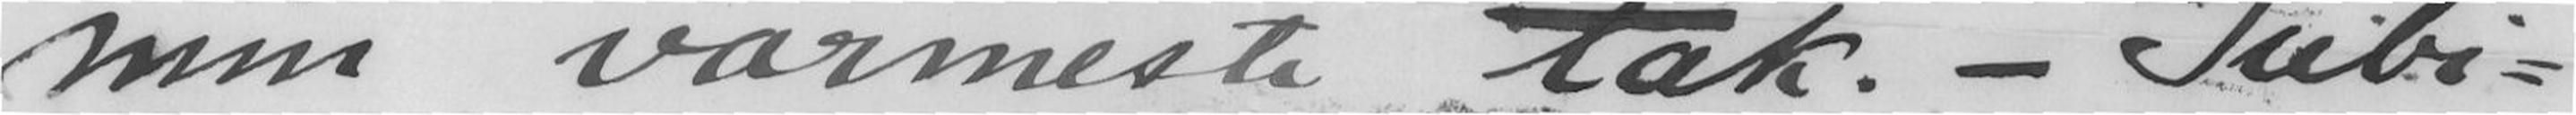min varmeste tak. - Jubi-
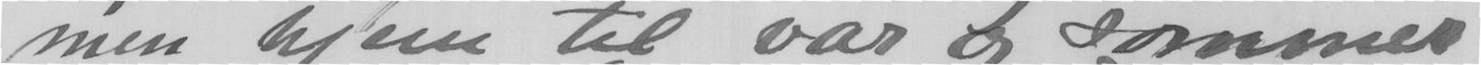men hjem til vår og sommer.
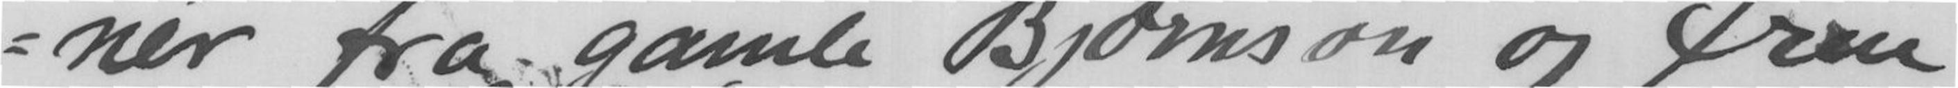=ner fra gamle Bjørnson og fra
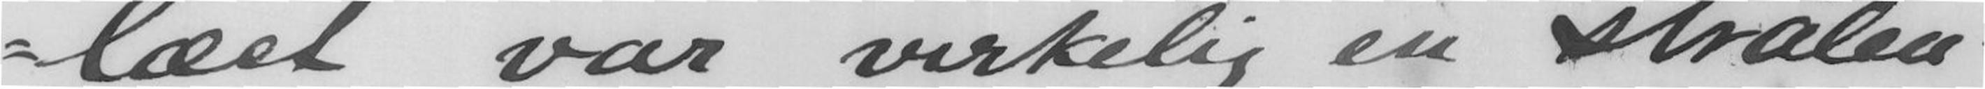læet var virkelig en strålen-
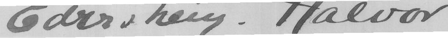Eders heng. Halvor
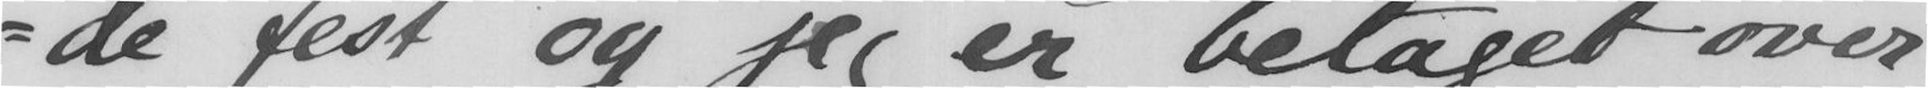de fest og jeg er betaget over
